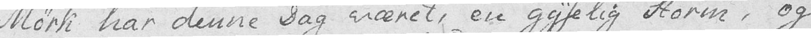Mørk har denne Dag været, en gyselig Storm, og
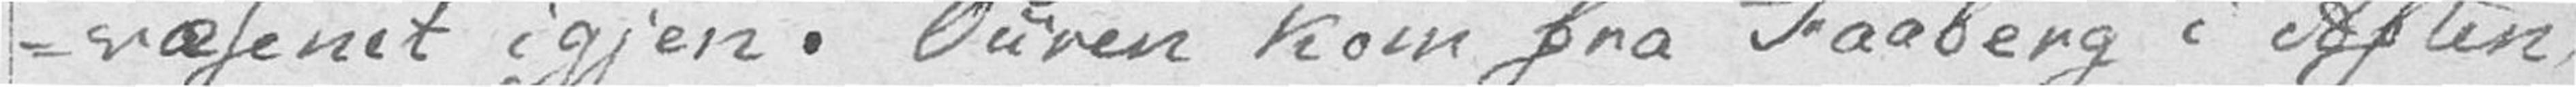-væsenet igjen. Ouren kom fra Faaberg i Aften,
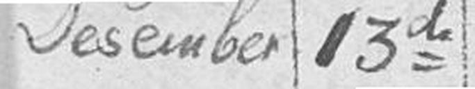Desember 13de
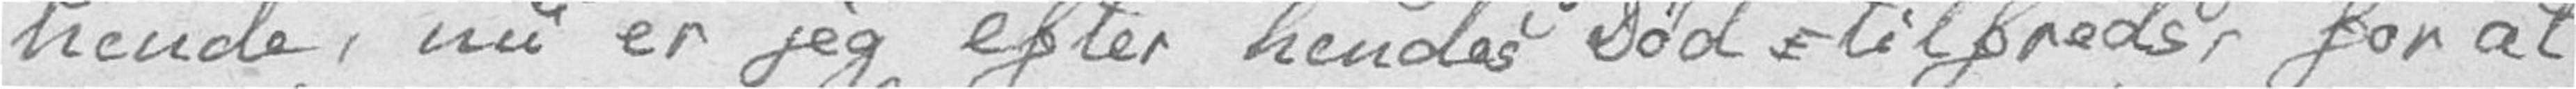hende, nu er jeg efter hendes Død tilfreds, for at
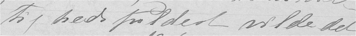tighedsfuldest vilde det
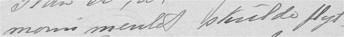monumentet skulde flyt-
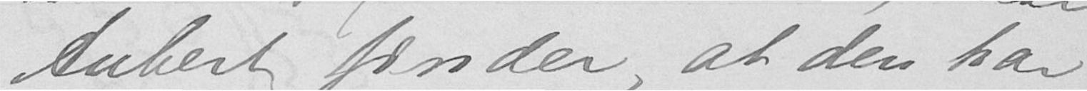Aubert finder, at den har
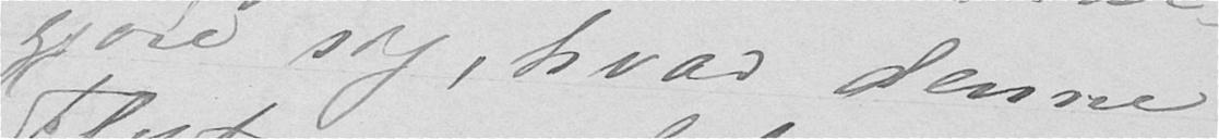gjøre sig, hvad denne
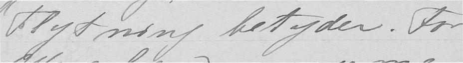Flytning betyder. For
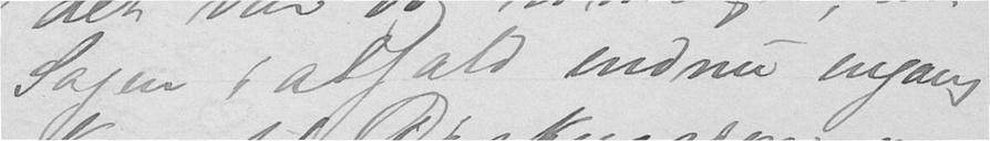Sagen i alfald endnu engang
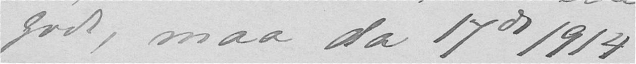godt, maa da 17de 1914
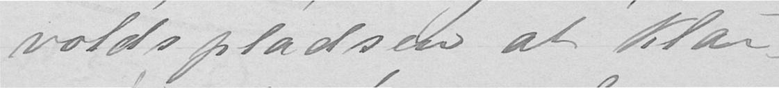voldspladsen at klar-
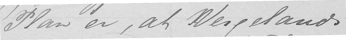Plan er, at Wergelands-
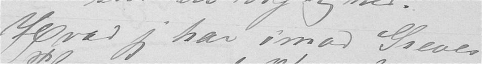Hvad jeg har imod Greves
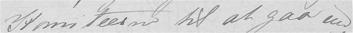Komiteen til at gaa med
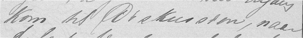kom til Diskussion, naar
